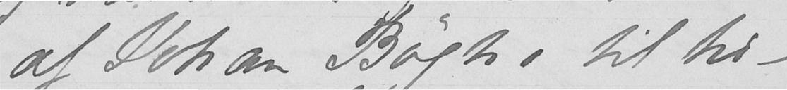af Johan Bøghs til hi-
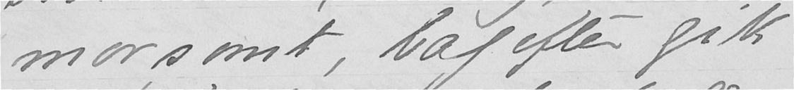morsomt, bagefter gik
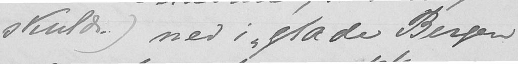skulde) ned i "glade Bergen"
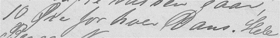10 Øre for hver Dans. Hele
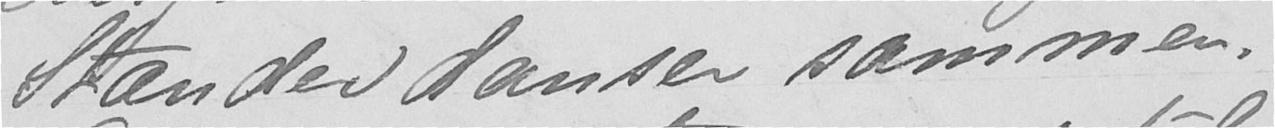Stænder danser sammen.
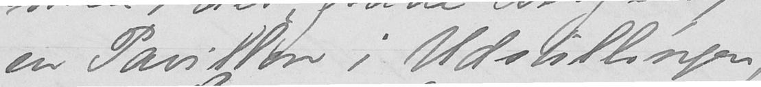en Pavillon i Udstillingen,
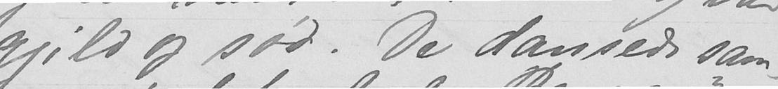Gjild og sød. De dansede sam-
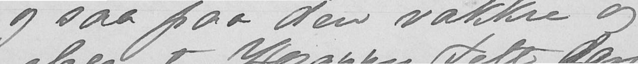og saa paa den vakkre og
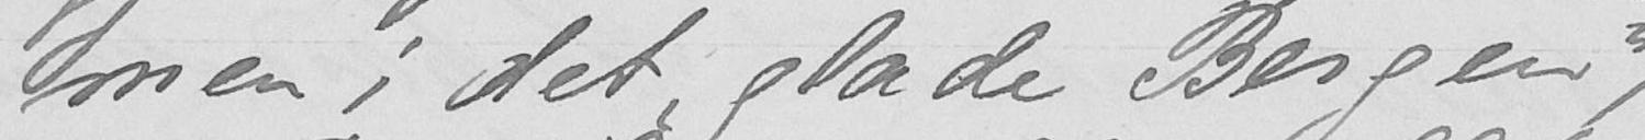men i det "glade Bergen",
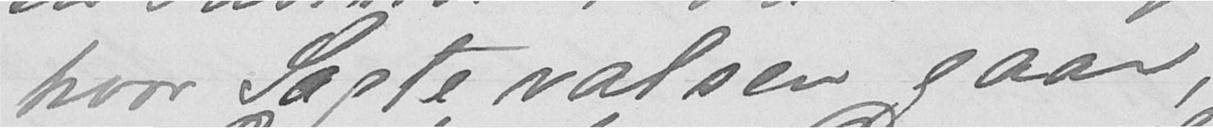hvor Sagtevalsen gaar,
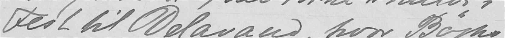Fest til Delavaud, hvor Bøghs
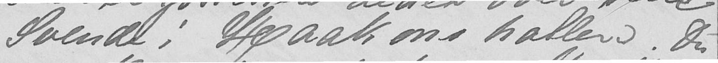Svende i Haakonshallen. Du
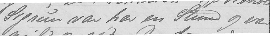Sigrun var her en Stund og var
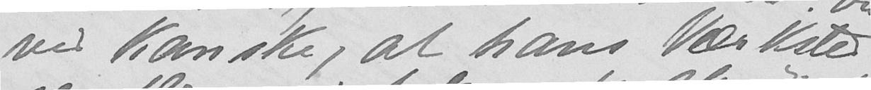ved kanske, at hans Værksted
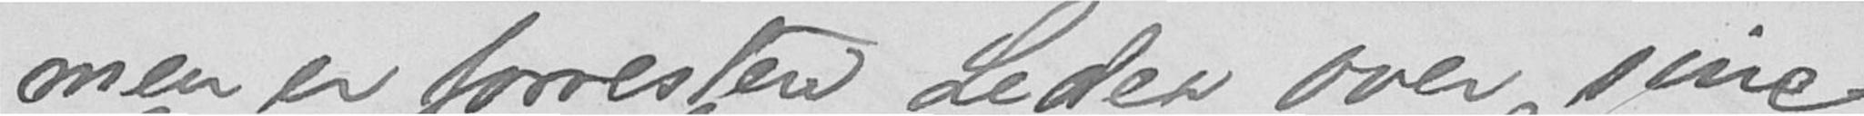men er forresten Leden over sine
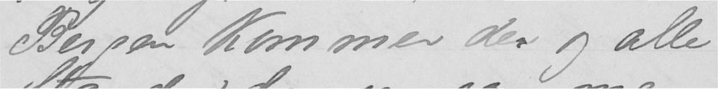Bergen kommer der og alle
